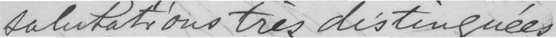salutations trés distinguées
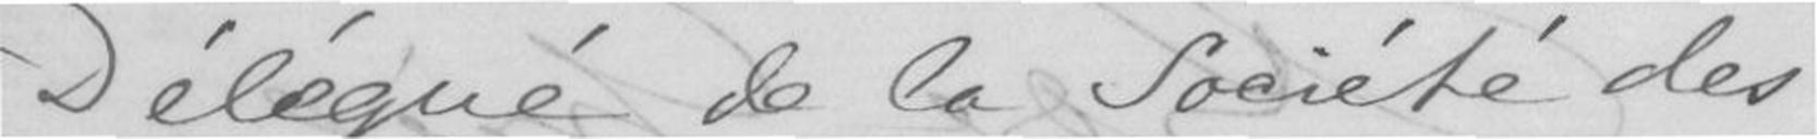Déléqué de la Société de
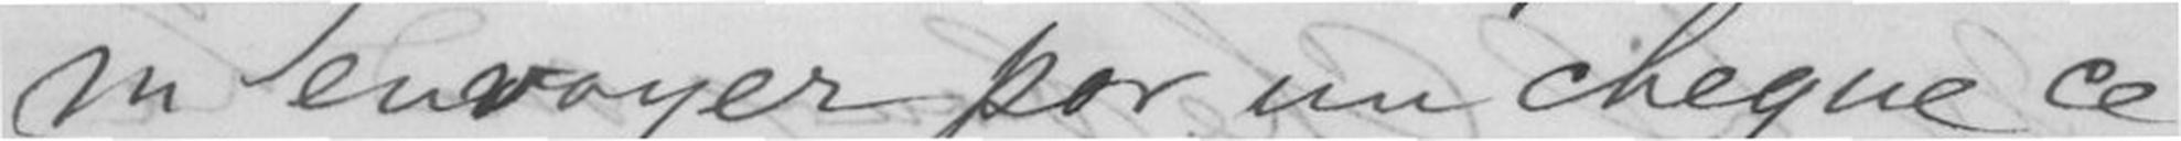ru envayer por un cheque ce
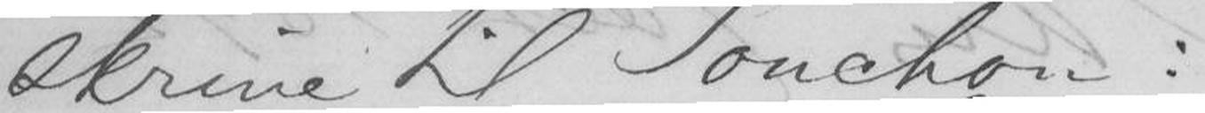skrive til Souchou:
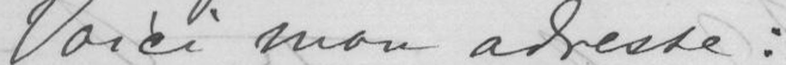Voici mon adresse:
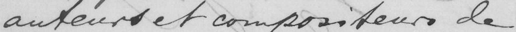auteurs et compositeurs de
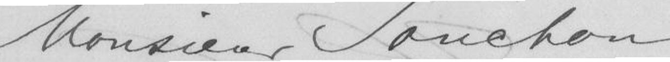Monsieur Soucheu
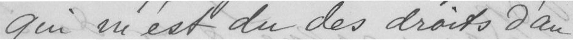qui ni'est du des droits d'au-
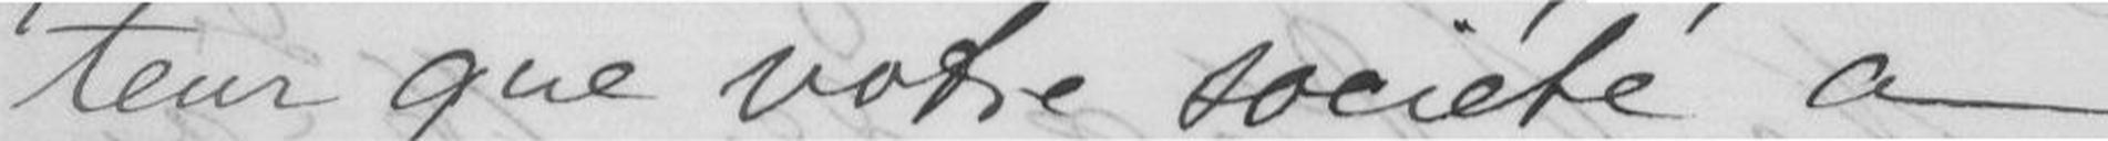teur que votre société a
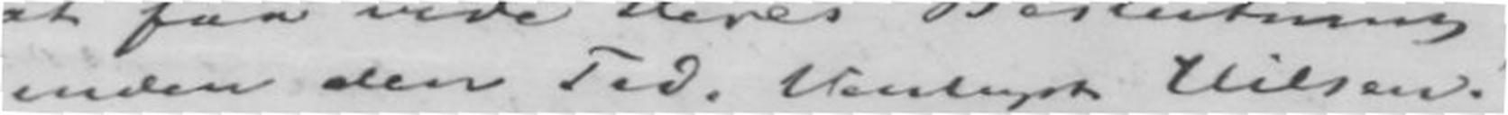inden den Tid. Venligst Hilsen!
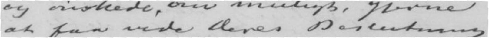at faa vide Deres Besentmann
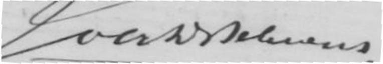Joh D Behrens
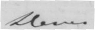Deres
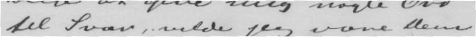til Svar, vilde jeg være Dem
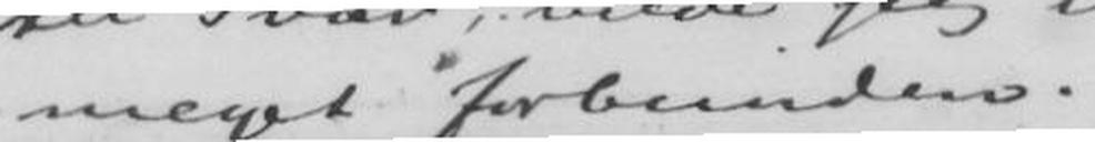meget forbunden.
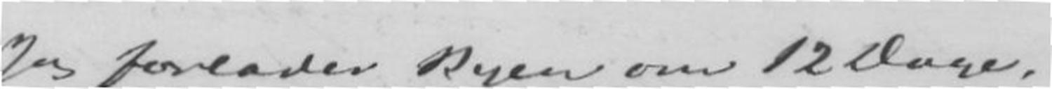Jeg forlader Byen om 12 Dage,
